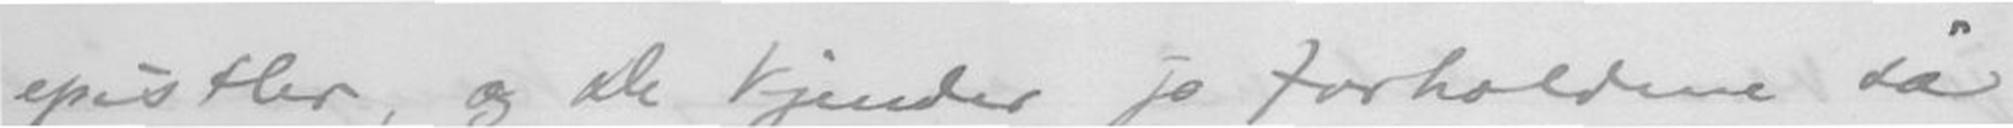epistler, og De kjender jo forholderne så
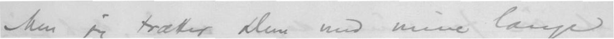Men jeg trætter Dem med mine lange
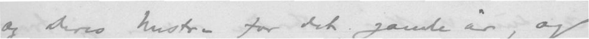og Deres hustru for det gamle år, og
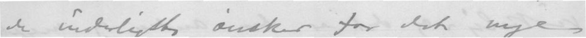de inderligste ønsker for det nye
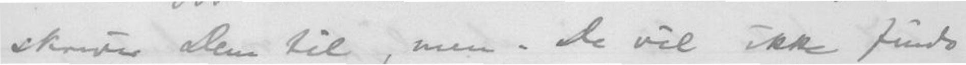skriver Dem til, men De vil ikke finde
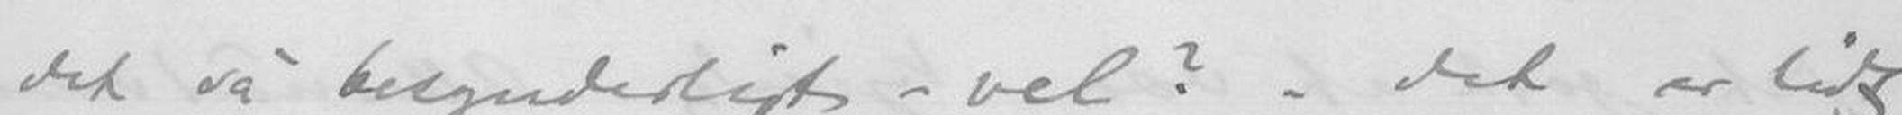det så besynderligt - vel? Det er lidt
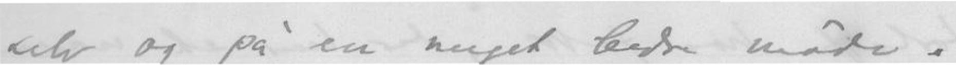selv og på en meget bedre måde.
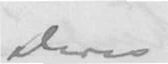Deres
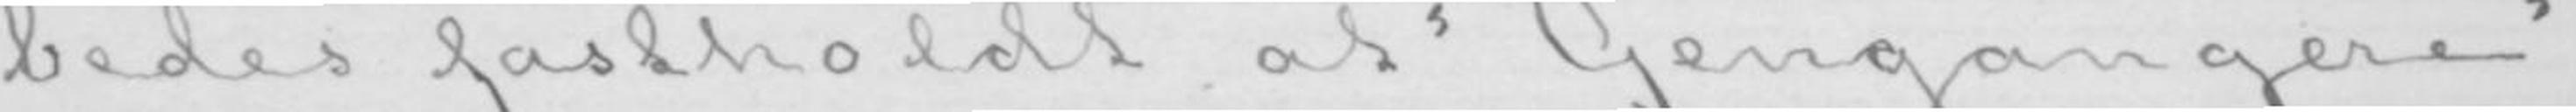bedes fastholdt at «Gengangere»,
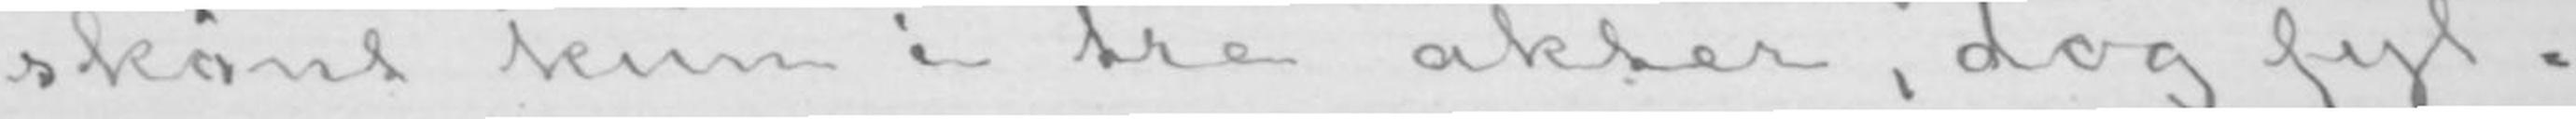skønt kun i tre akter, dog fyl-
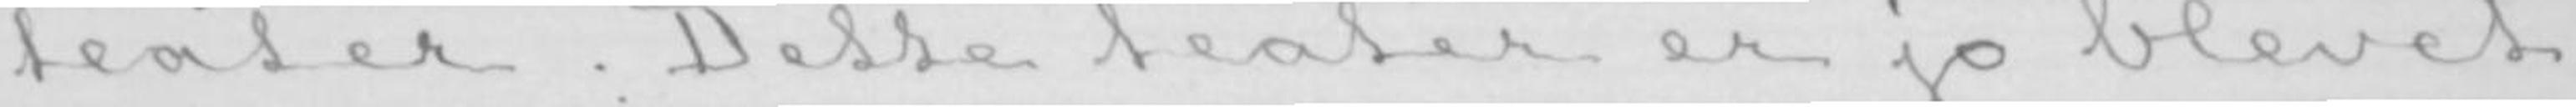teater. Dette teater er jo blevet
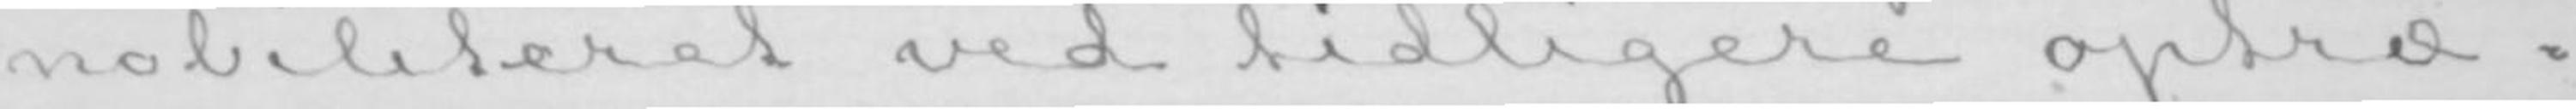nobiliteret ved tidligere optræ-
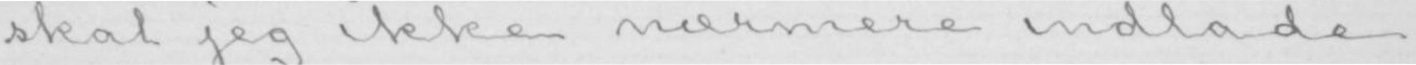skal jeg ikke nærmere indlade
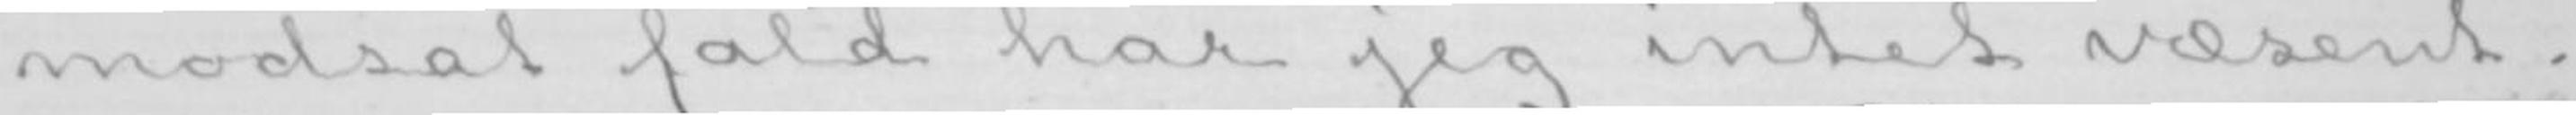modsat fald har jeg intet væsent-
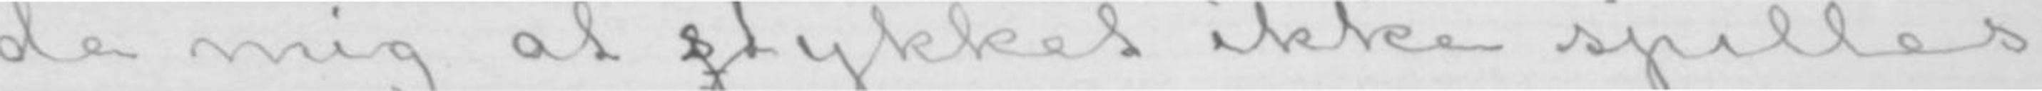de mig at stykket ikke spilles
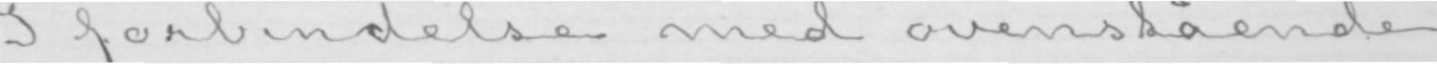I forbindelse med ovenstående
